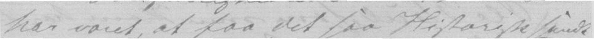har været, at faa det saa Historisk sandt
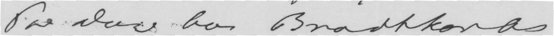Par Dage hos Brodtkovses
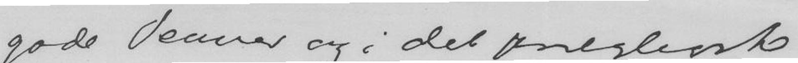gode Venner og i det prægtigske
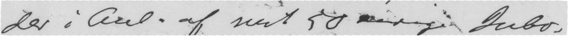der i Anl. af mit 50 aarige Jubi-
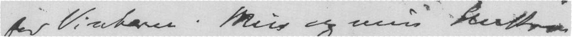for Vinteren. Min og min Hustrus
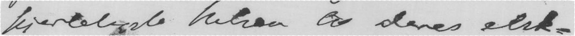hjerteligste hilsen til Deres elsk-
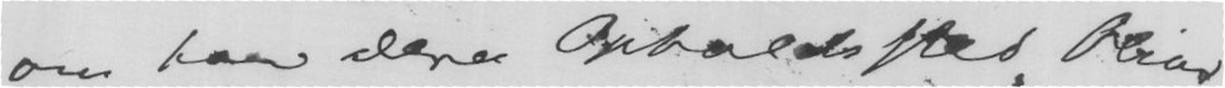om hva Deres Opholdssted bliver
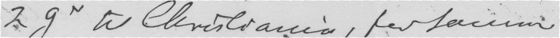29de til Christiania, for samme
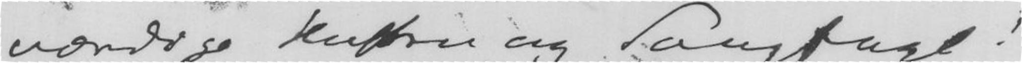værdige Hustru og Sangfugl!
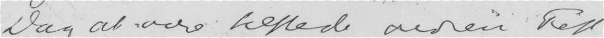Dag at være tilstede ved en Fest
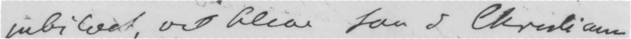jubilæet, vil blive som i Christiania
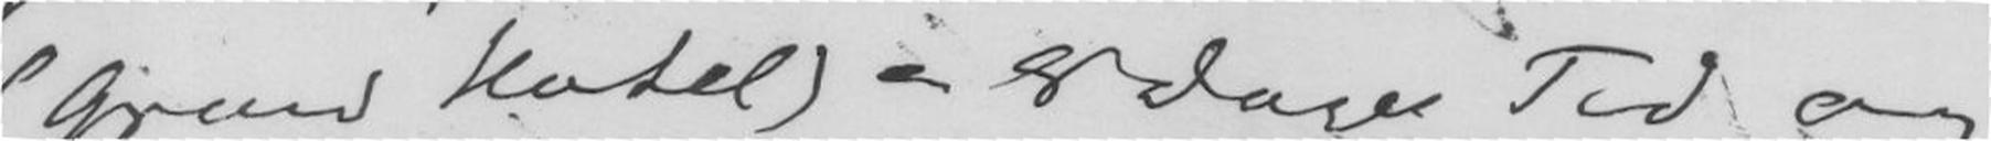(Grand Hotel) i 8 dages Tid og
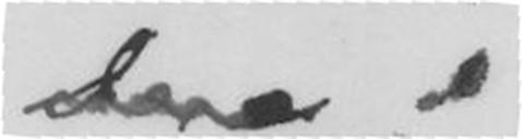Deres
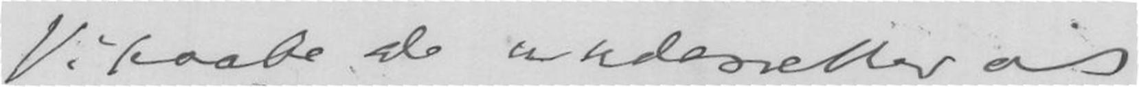Vi haabe De underretter os
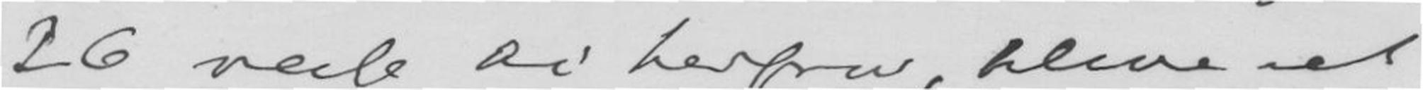26 reise vi herfra, blive et
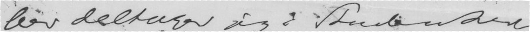ber deltager jeg i Studenter-
